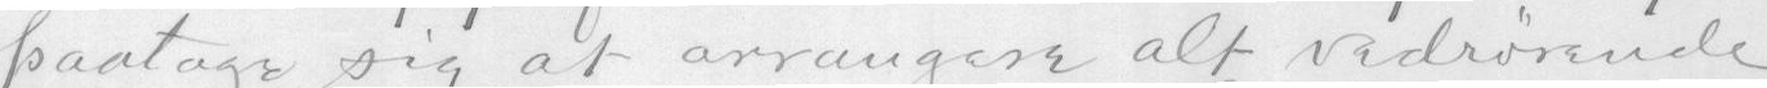paatage sig at arrangere alt vedrørende
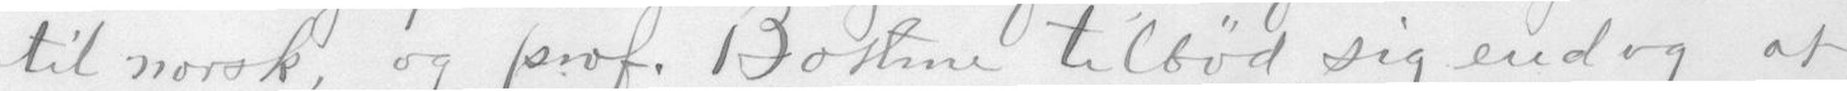til norsk, og prof. Bothne tilbød sig endog at
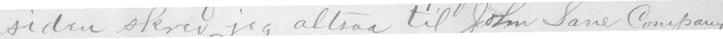siden skrev jeg altsaa til John Save Company
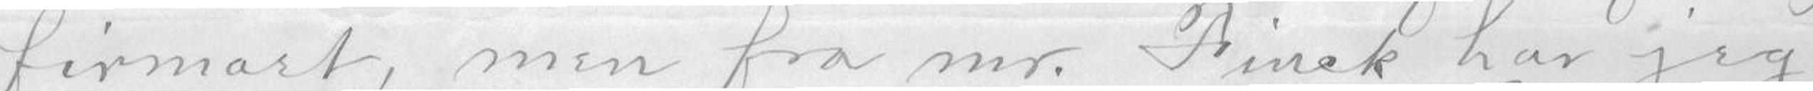firmaet, men fra mig. Finck har jeg
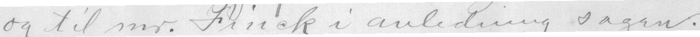og til mr. Finck i anledning sagan.
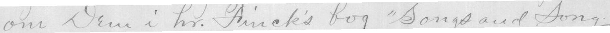om Dem i hr. Finck's bog "Songs and Song
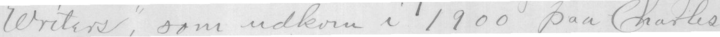Writers", som udkom i 1900 paa Charles
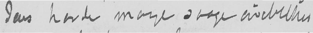Jens havde mange svage øyeblikk
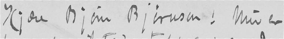Kjære Bjørn Bjørnson! Nu er
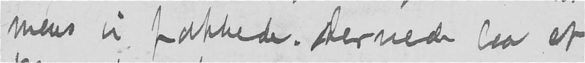mens vi pakkede. dernede laa et
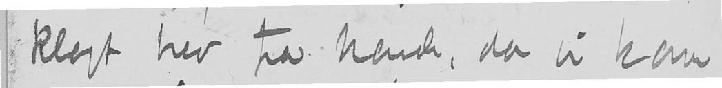klogt brev fra hende, da vi kom
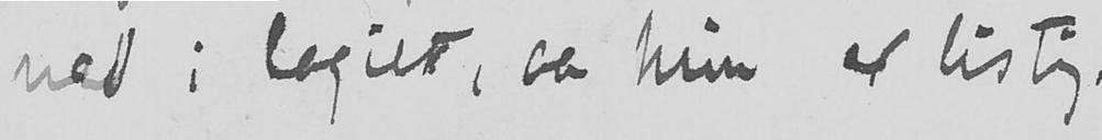ned i logiet, aa hun er listig.
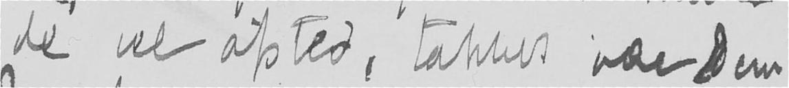de vel afsted, takket være dem.
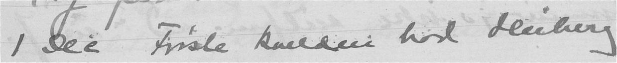1 Dec Første kvelden bad Heiberg
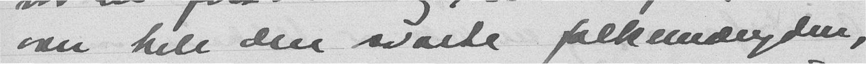over hele den svarte folkemængden,
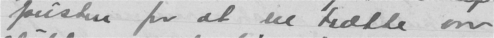pusten for at en trætte var
